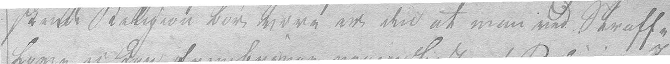skende Religion bør være er den at man ved Straffe-
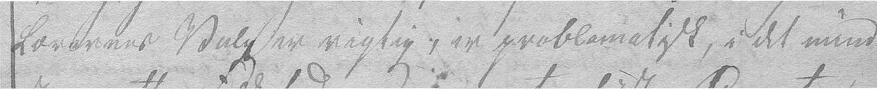Lærernes Valg er rigtig, er problematisk, i det mind-
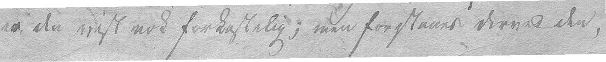er den vist nok forkastelig; men forstaaes derved den,
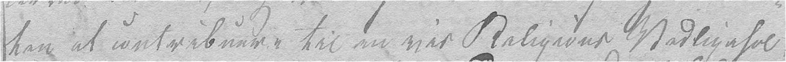ten at contribuere til en vis Religions Vedligehol-
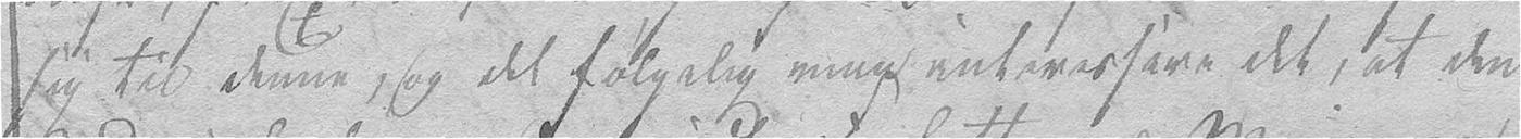sig til denne, og det følgelig maa interessere det, at den
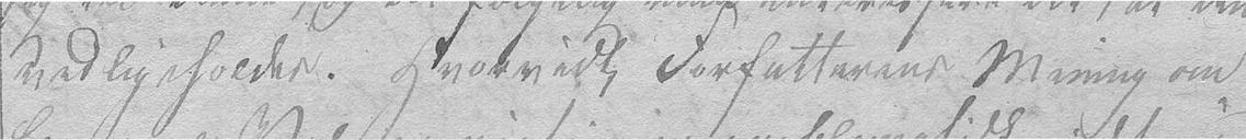vedligeholdes. Hvorvidt Forfatterens Mening om
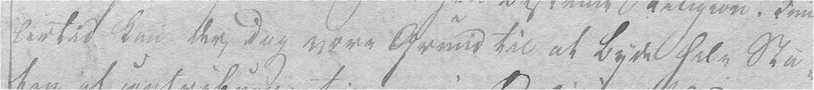lertid kan der dog være Grund til at byde hele Sta-
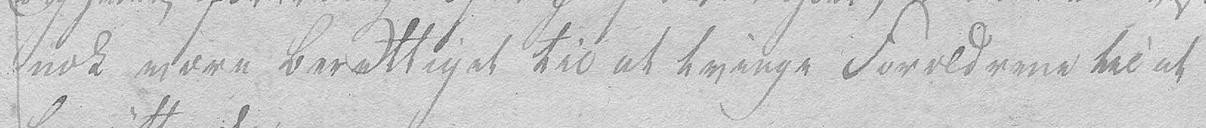nok være berettiget til at tvinge Forældrene til at
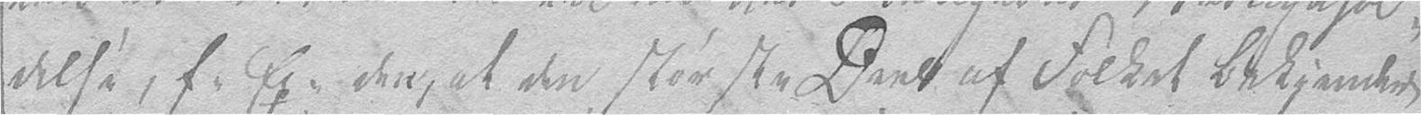delse, f: Ex: den, at den største Deel af Folket bekjender
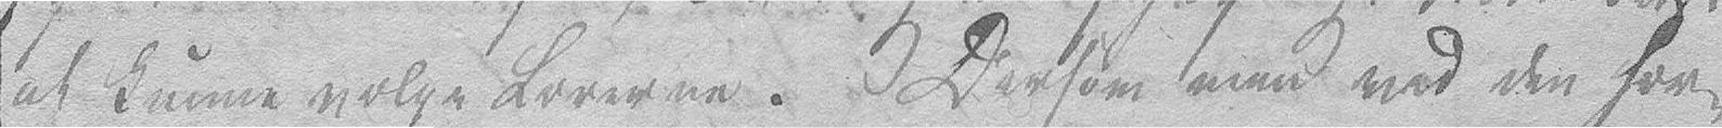at kunne vælge Lærerne. Dersom man ved den her-
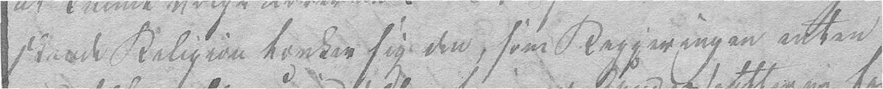skende Religion tænker sig den, som Regjeringen enten
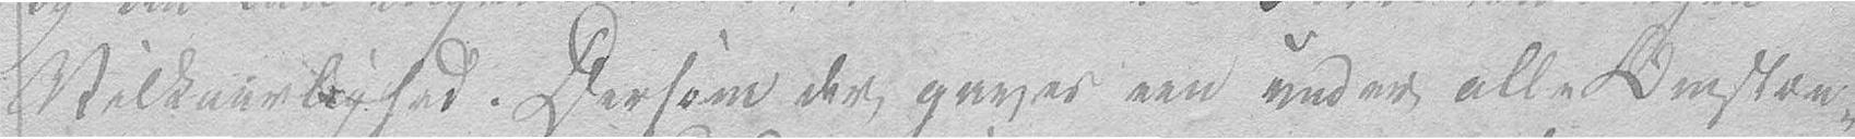Vilkaarlighed. Dersom der gaves een under alle Omstæn-
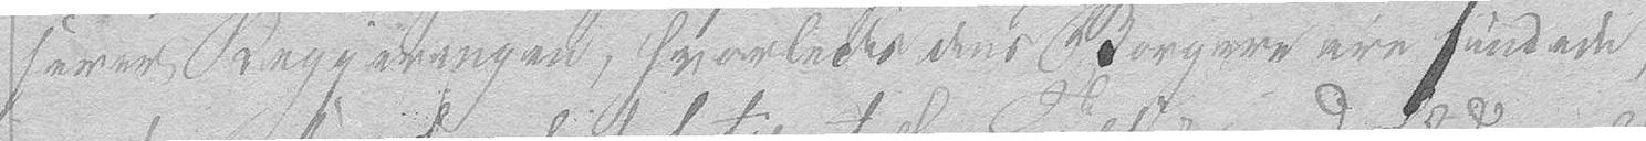serer Regjeringen, hvorledes dens Borgere ere fundede,
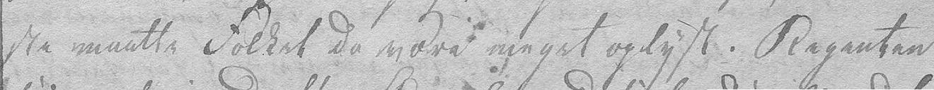ste maatte Folket da være meget oplyst. Regenten
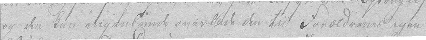og den kan ingenlunde overlade den til Forældrenes egen
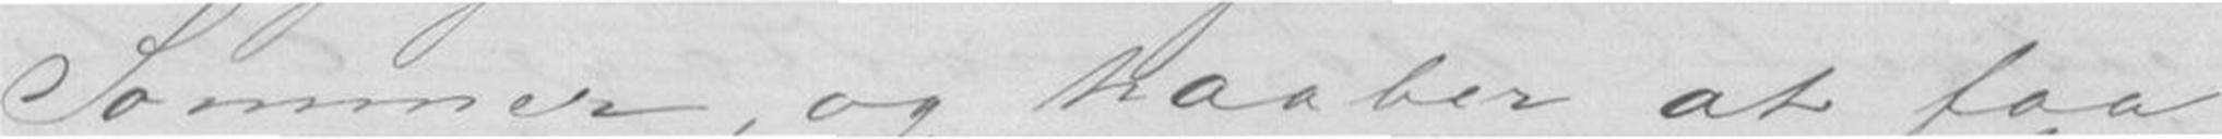Sommer, og haaber at faa
